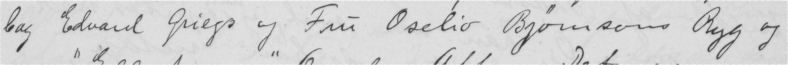bag Edvard Griegs og Fru Oselio Bjørnsons Ryg og
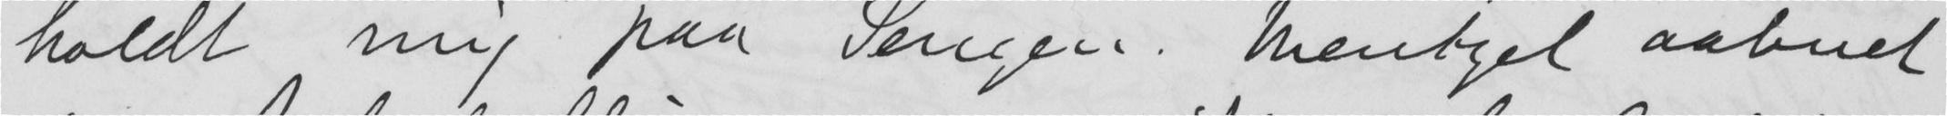holdt mig paa Sengen. Wentzel aabnet
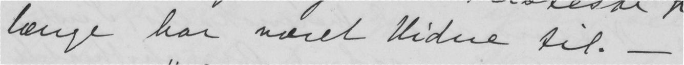længe har været Vidne til. —
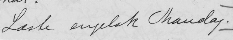Læste engelsk Mandag.
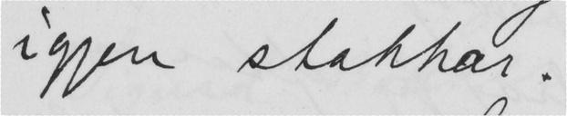igjen stakkar.
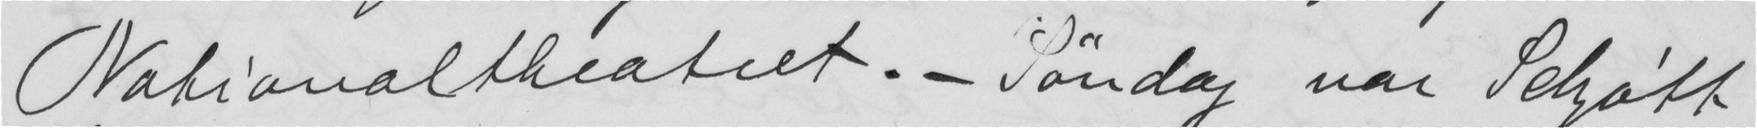Nationaltheatret. - Søndag var Schjøtt,
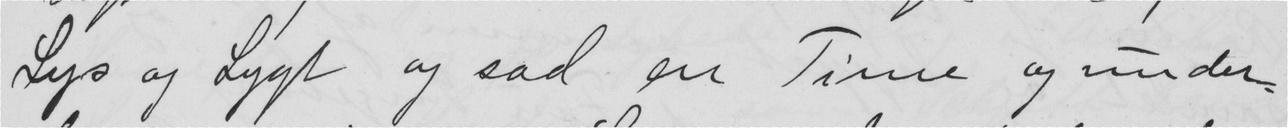Lys og Lygt og sad en Time og under-
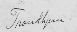Trondhjem.
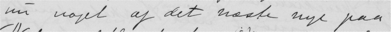nu noget af det næste nye paa
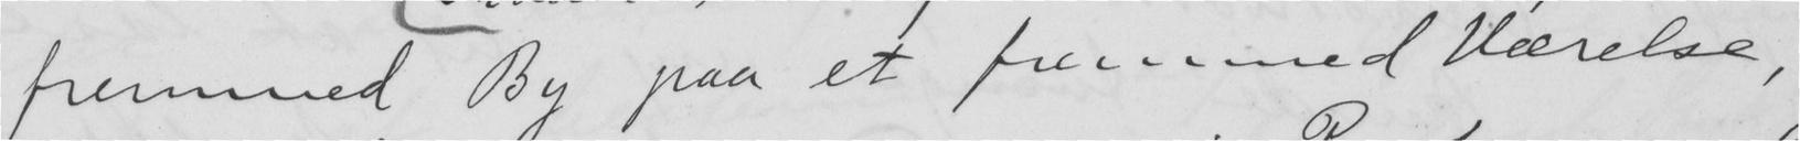fremmed By paa et fremmed Værelse,
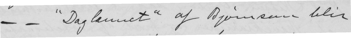- - "Daglannet" af Bjørnson blir
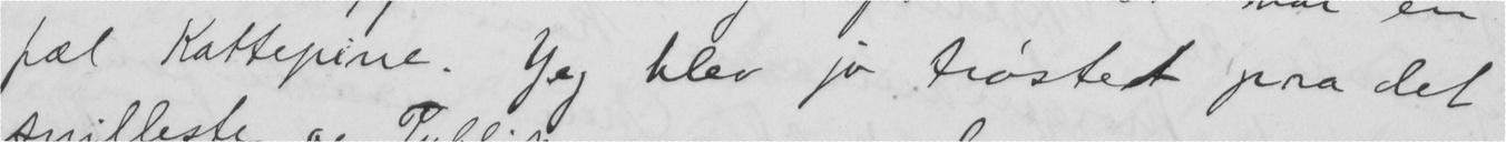fæl Kattepine. Jeg blev jo trøstet pra det
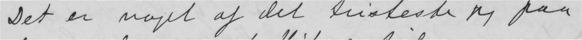Det er noget af det tristeste jeg paa
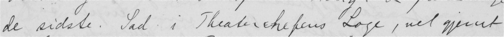de sidste. Sad i Theaterchefens Loge, vel gjemt
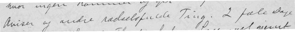aviser og andre rædselsfulde Ting. 2 fæle Dage
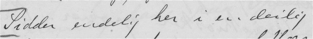Sidder endelig her i en deilig
